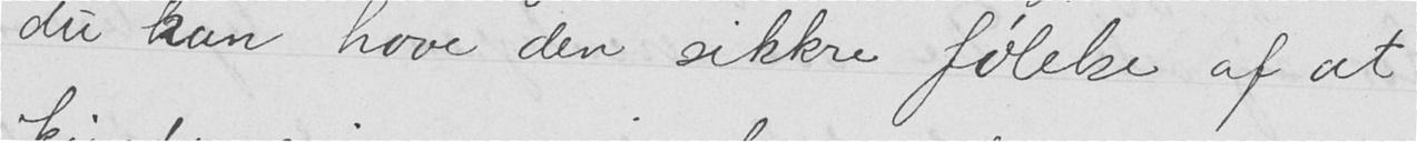du kan have den sikkre følelse af at
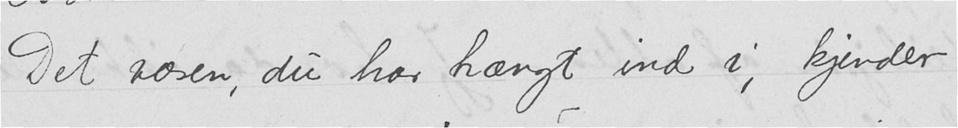Det væsen, du har trængt ind i, kjender
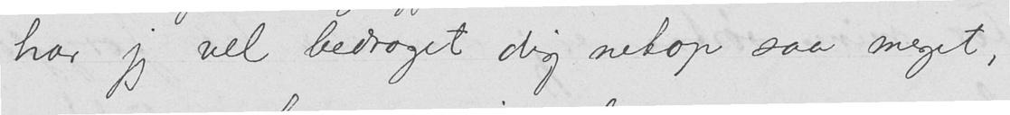har jeg vel bedraget dig netop saa meget,
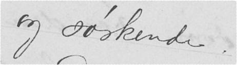og søskende.
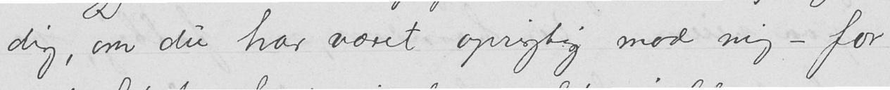dig, om du har været oprigtig mod mig - for
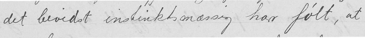det bevidst instinktsmæssig har følt, at
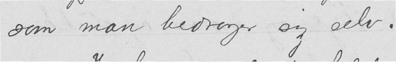som man bedrager sig selv.
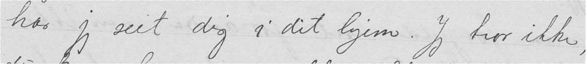har jeg seet dig i dit hjem. Jeg tror ikke,
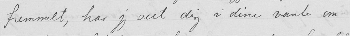fremmet, har jeg seet dig i dine vante om-
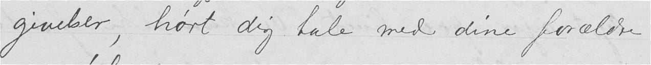givelser, hørt dig tale med dine forældre
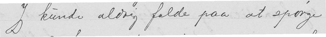Jeg kunde aldrig falde paa at spørge
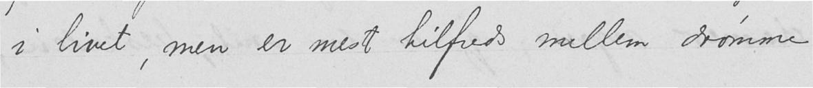i livet, men er mest tilfreds mellem drømme
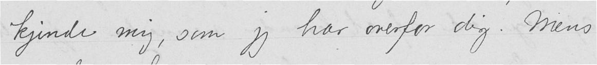kjende mig, som jeg har overfor dig. Mens
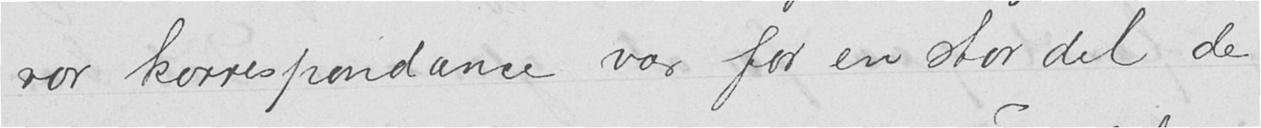vor korrespondance var for en stor del de
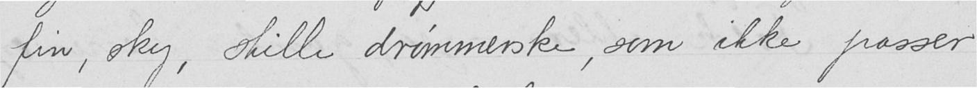fin, sky, stille drømmerske, som ikke passer
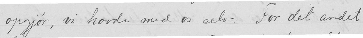opgjør, vi havde med os selv. For det andet
